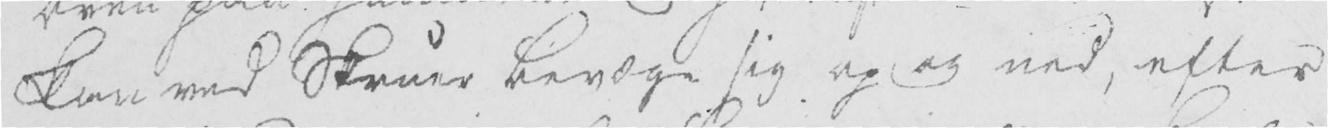kun ved Skruer bevæge sig op og ned, efter
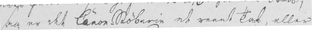saa er det Canon-Støberie et reent Tab, eller
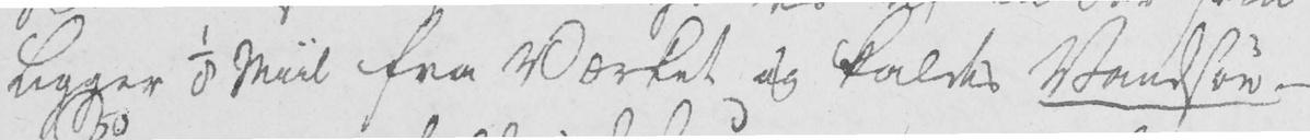ligger 1/8 Miil fra Værket og kaldes Vandsøe -
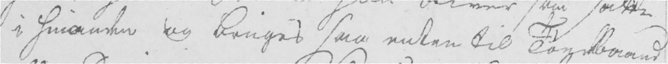i hinanden og bruges saa enten til Tøndbaand
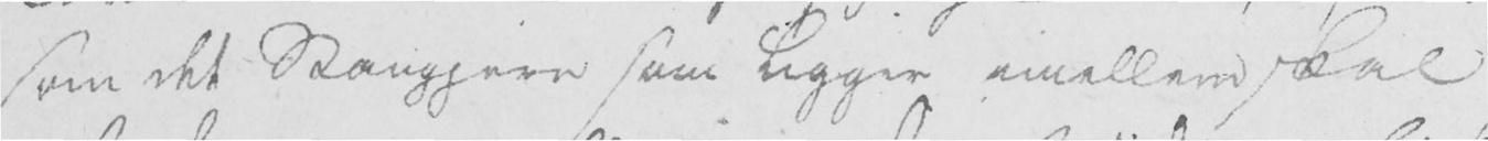som det Stangjern som ligger imellem skal
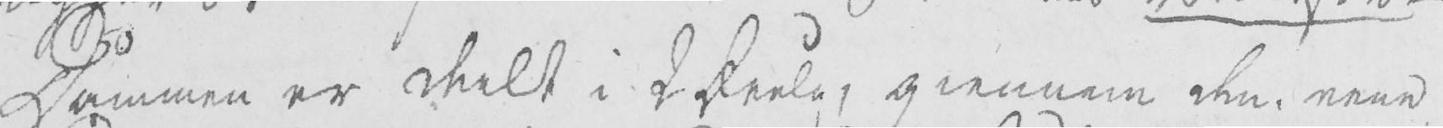Dammen er deelt i 2 Deele, giennem den eene
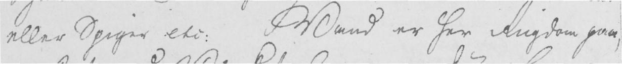eller Spiger etc. Vand er her Riigdom paa,
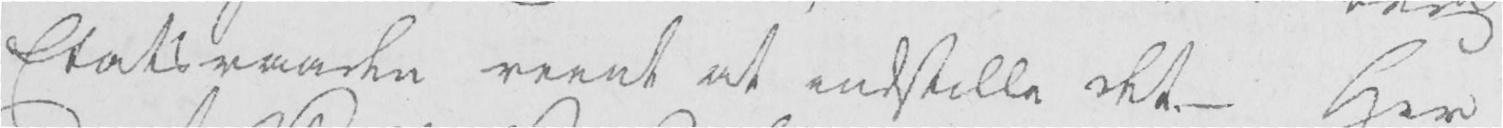Etatsraaden reent at indstille det. - Her
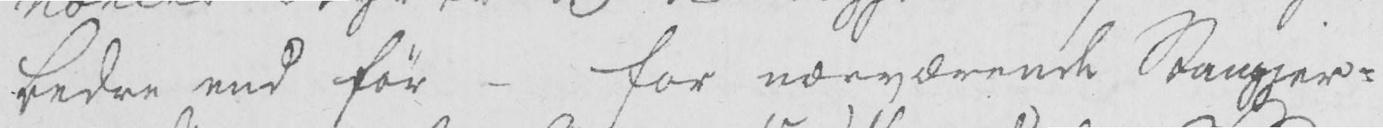bedre end før - for nærværende Stangjer-
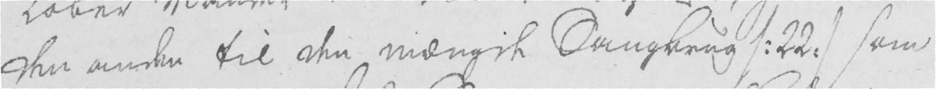den anden til den mængde Saugbrug /: 22 :/ som
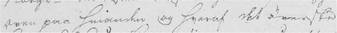oven paa hinanden og hvoraf det øverste
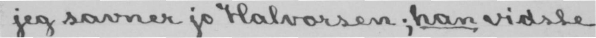jeg savner jo Halvorsen; han vidste
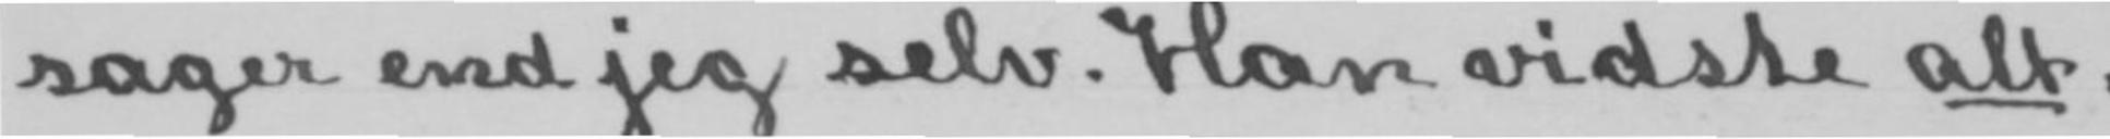sager end jeg selv. Han vidste alt.
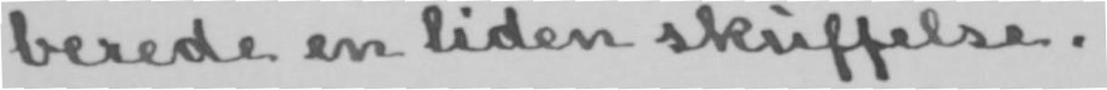berede en liden skuffelse.
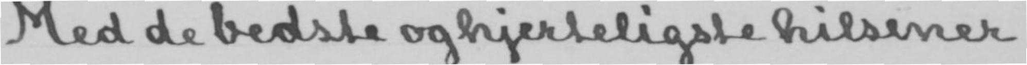Med de bedste og hjerteligste hilsener
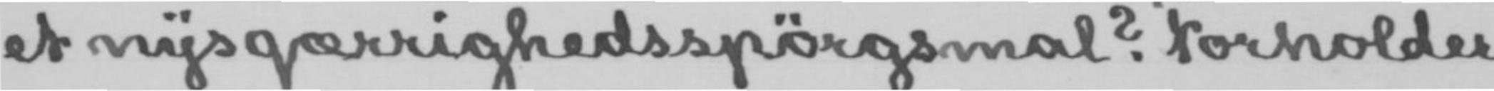et nysgærrighedsspørgsmål? Forholder
